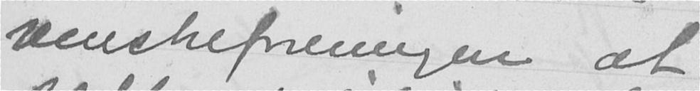venstreforeningen at
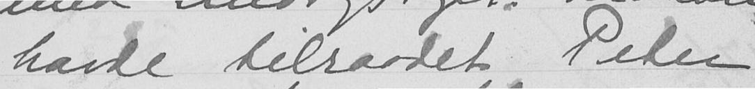havde tilraadet Peter
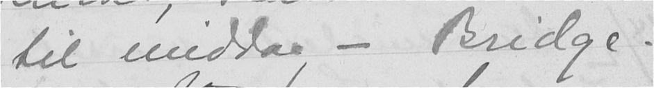til middag - Bridge.
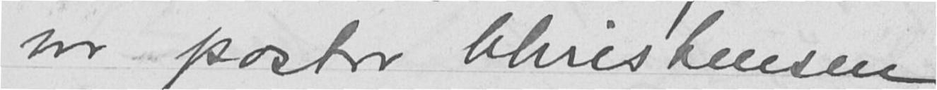var pastor Christensen
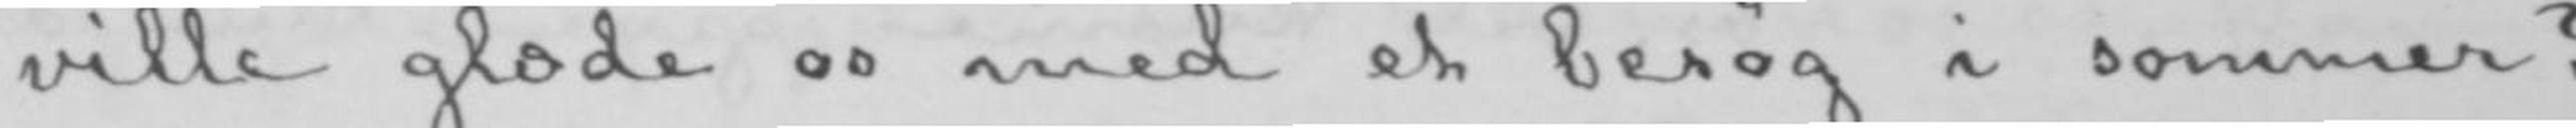ville glæde os med et besøg i sommer?
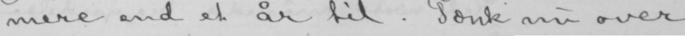mere end et år til. Tænk nu over
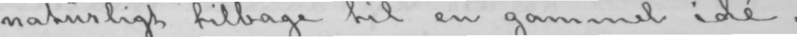naturligt tilbage til en gammel idé.
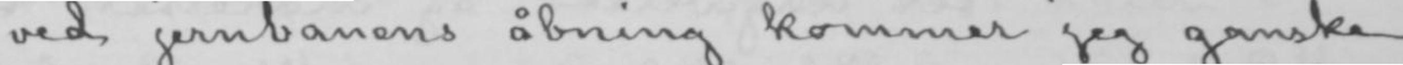ved jernbanens åbning kommer jeg ganske
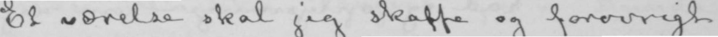Et værelse skal jeg skaffe og forøvrigt
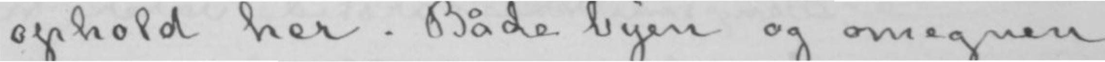ophold her. Både byen og omegnen
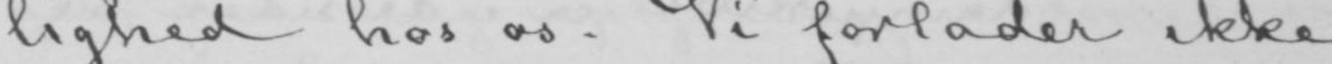lighed hos os. Vi forlader ikke
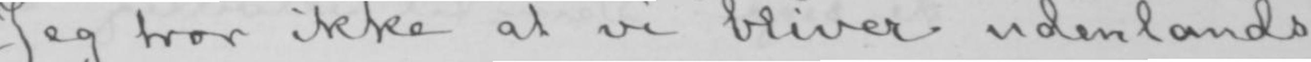Jeg tror ikke at vi bliver udenlands
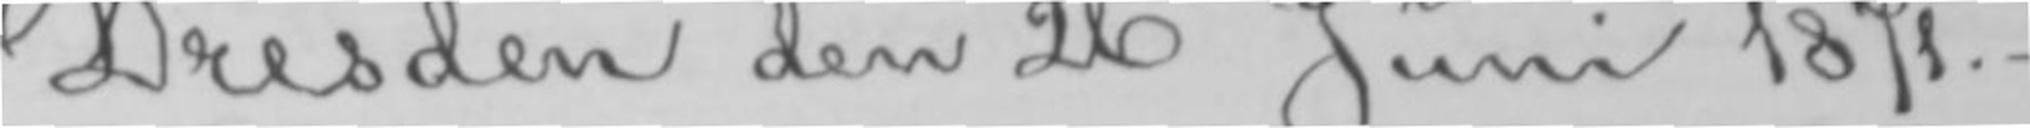Dresden den 26de Juni 1871.-
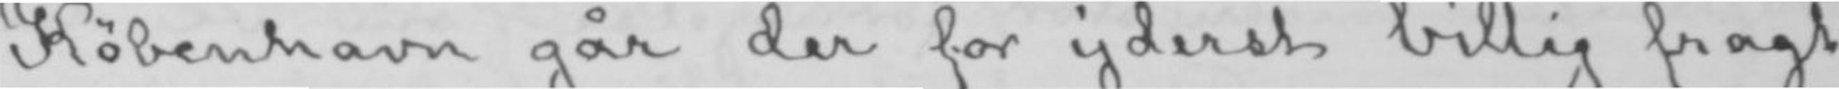København går der for yderst billig fragt
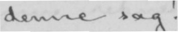denne sag!
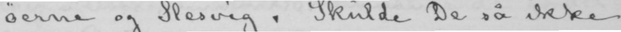øerne og Slesvig. Skulde De så ikke
